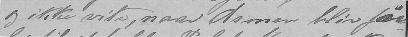og ikke vite, naar Armen blir pas-
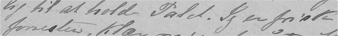lig til at holde Palet. Jeg er frisk
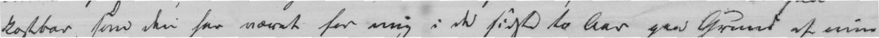kostbar, som den har været for mig i det sidste to Aar paa Grund af min
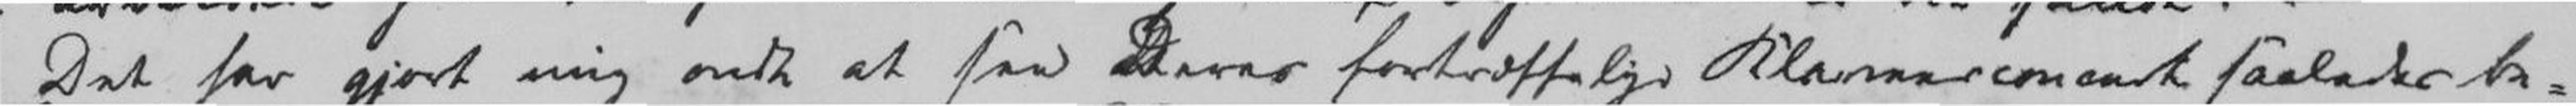Der har gjort mig ondt at see Deres fortræffelige Klaveerconcerte saaledes be-
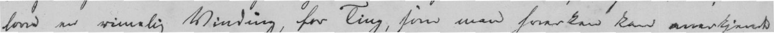love en rimlig Vinding, for Ting, som man hverken kan anerkjende
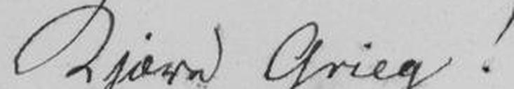Kjære Grieg!
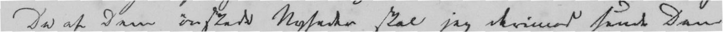De af Dem ønskede Nyheder skal jeg derimod sende Dem
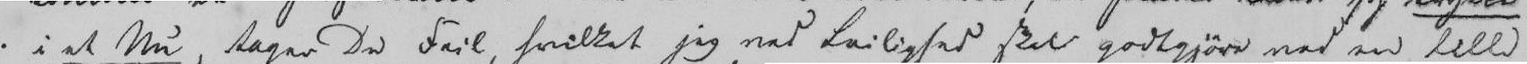i et Nu, tager De Feil, hvilket jeg ved Leilighed skal godtgjøre ved en lille
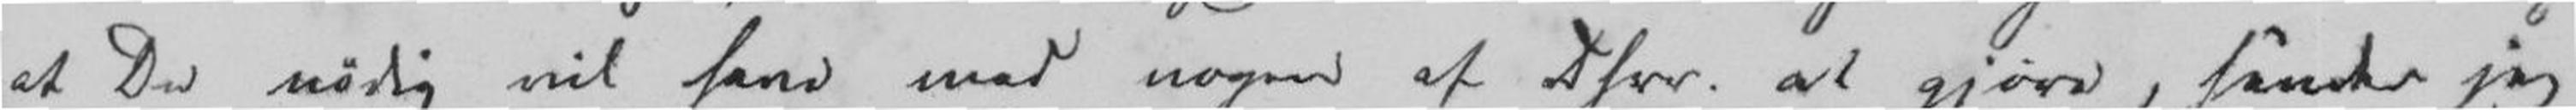at De nødig vil have med nogen af Dhrr. at gjøre, sender jeg
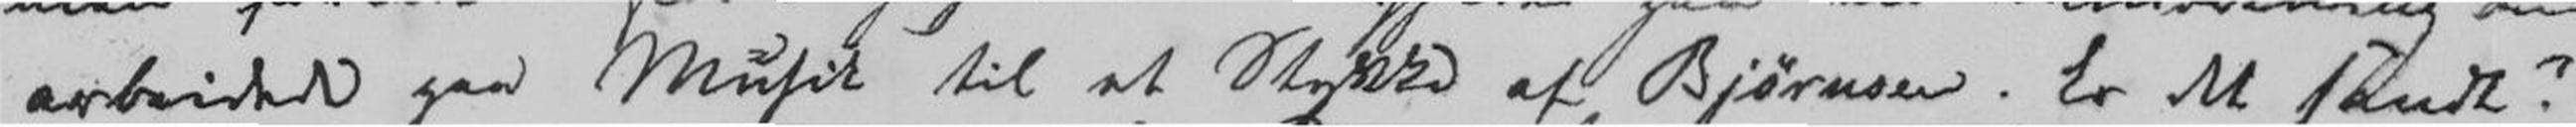arbeidet paa Musik til et Stykke af Bjørnsen. Er det Sandt? -
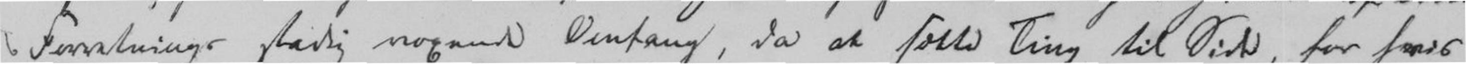Forretnings stadig voxende Omfang, da at sætte Ting til Side, for hans
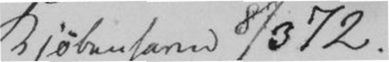Kjøbenhavn 8/3 72
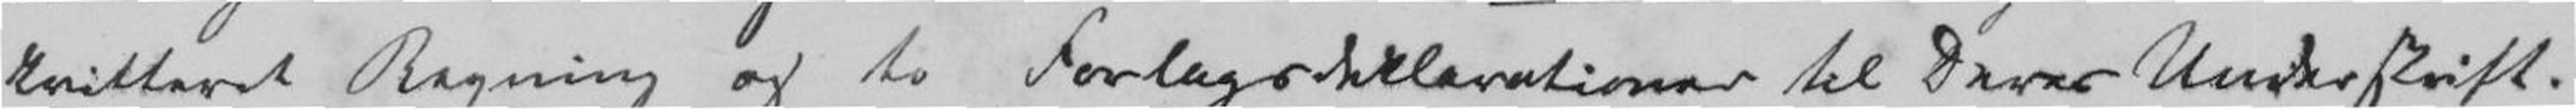kviteret Regning og to Forlægsdeklarationer til Deres Underskrift. -
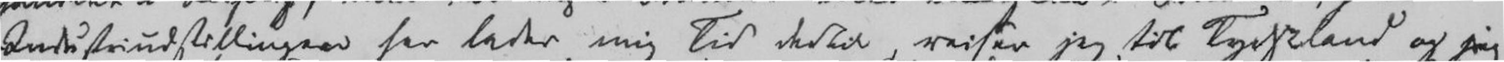Industriudstillengen her lader mig Tid dertil reiser jeg til Tydskland og jeg
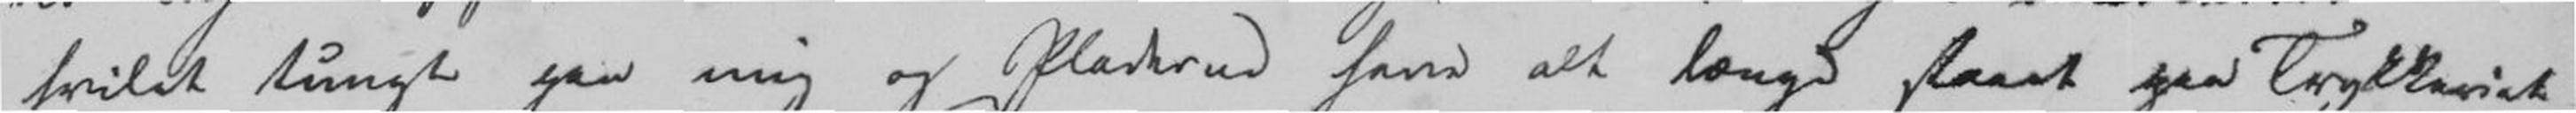hvilet tungt paa mig og Pladerne have alt længe staaet paa Trykkeriet
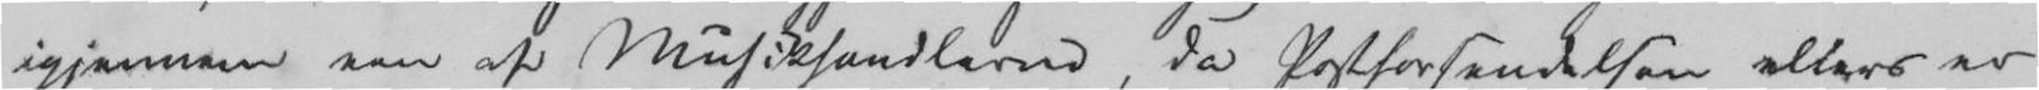igjennem een af Musikhandlerne, da Postforsendelsen ellers er
